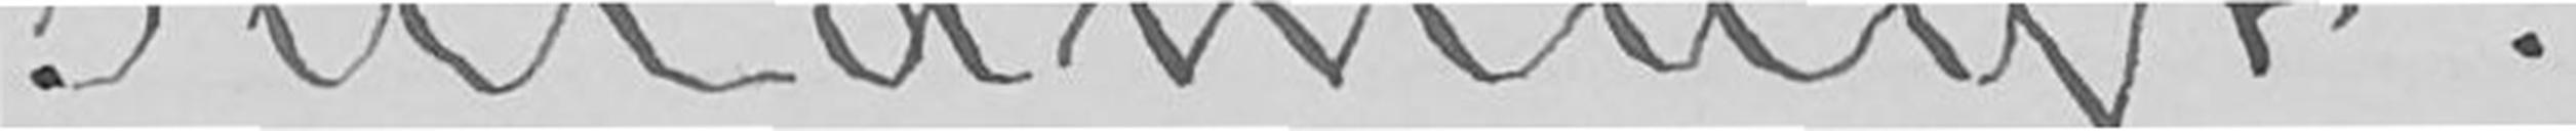Fuldmagt.
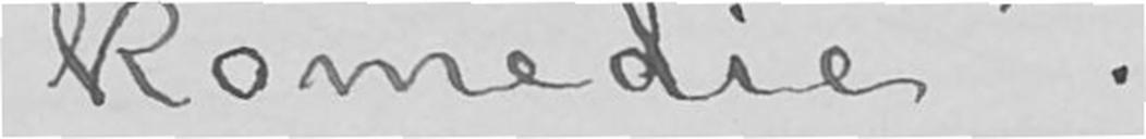Komedie».
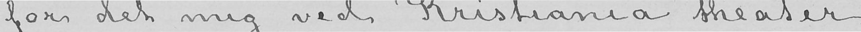for det mig ved Kristiania theater
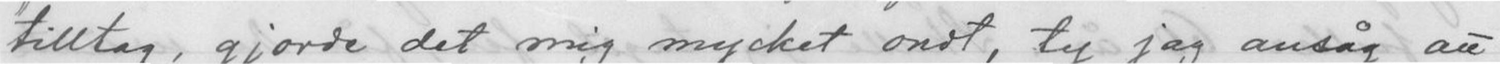tilltag, gjorde det mig mycket ondt, ty jag ansåg att
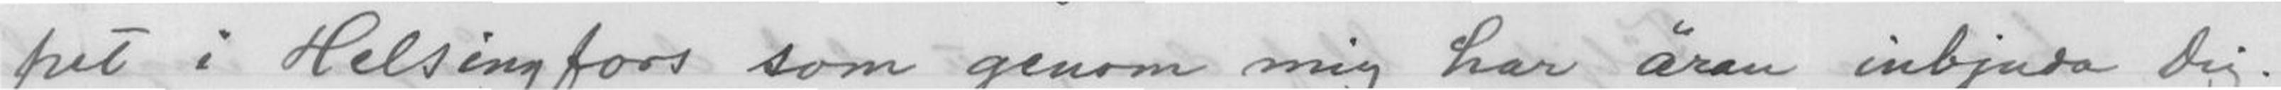pet i Helsingfors som genom mig har äran inbjuda Dig.
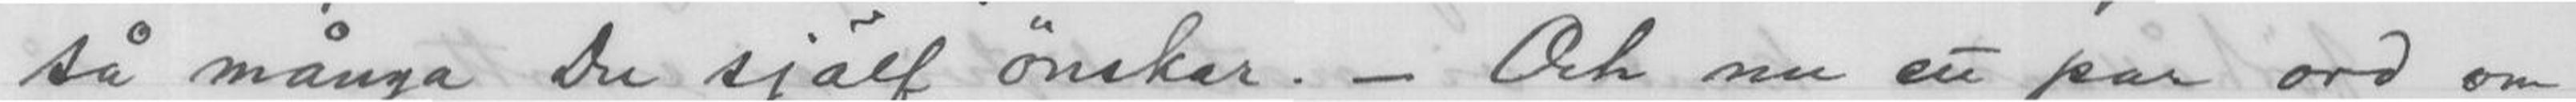så många Du själf önskar. - Och nu ett par ord om
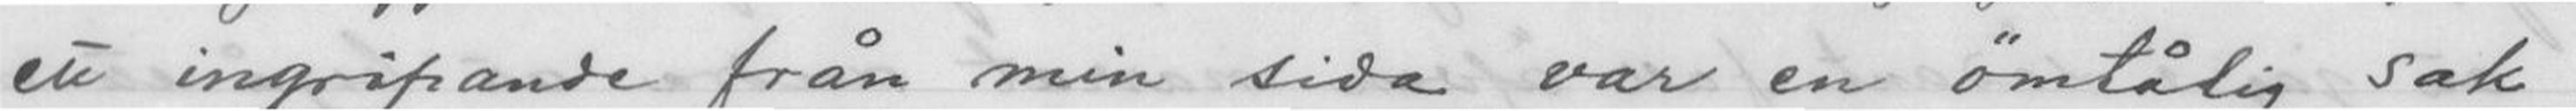ett ingripande från min sida var en ömtålig sak,
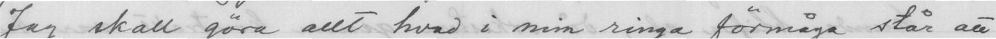Jag skall göra allt hvad i min ringa förmåga står att
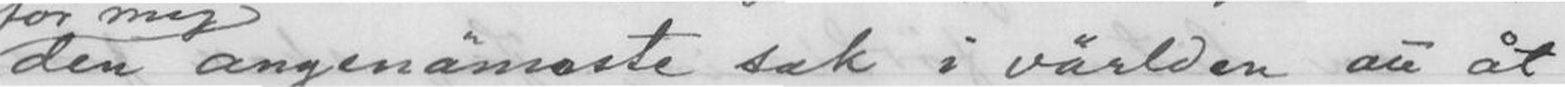den angenämesta sak i världen att åt
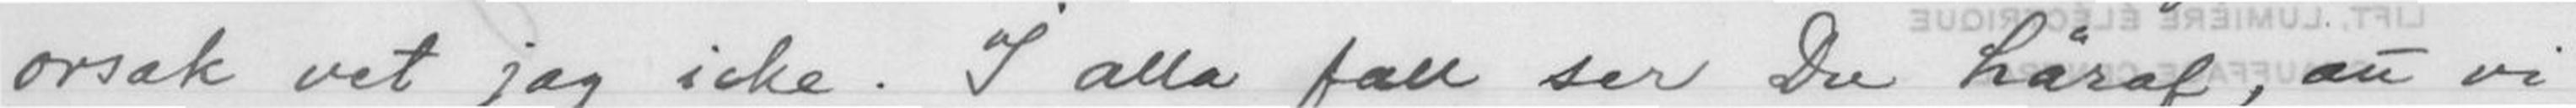orsak vet jag icke. I alla fall der Du häraf, att vi
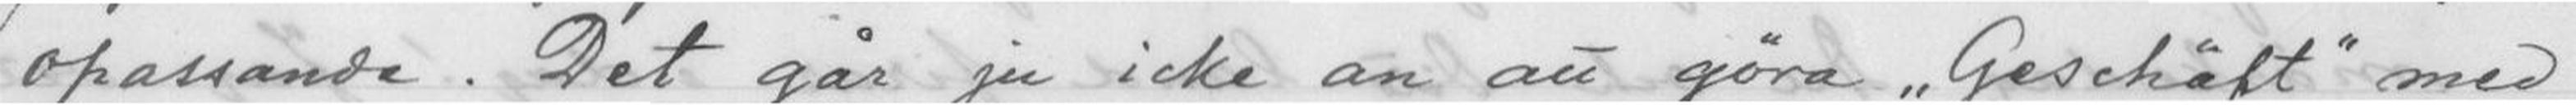opassande. Det går ju icke an att göra Geschäft med
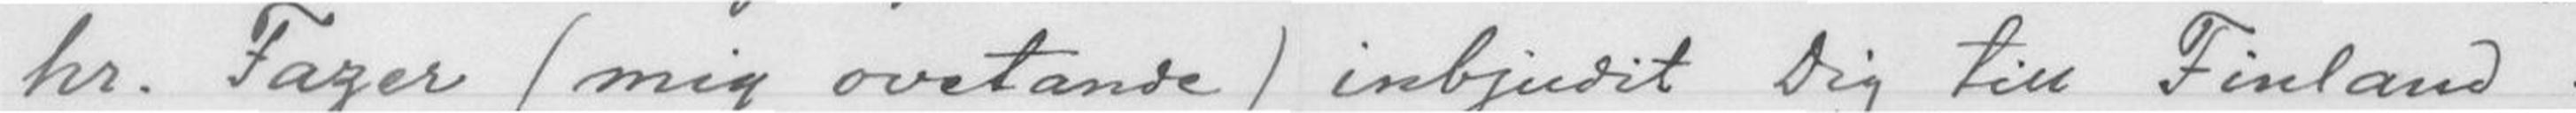hr. Fager (mig ovetande) inbjudit Dig till Finland.
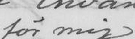för mig
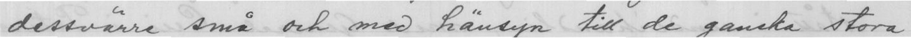dessvärre små och med hänsyn till de ganske stora
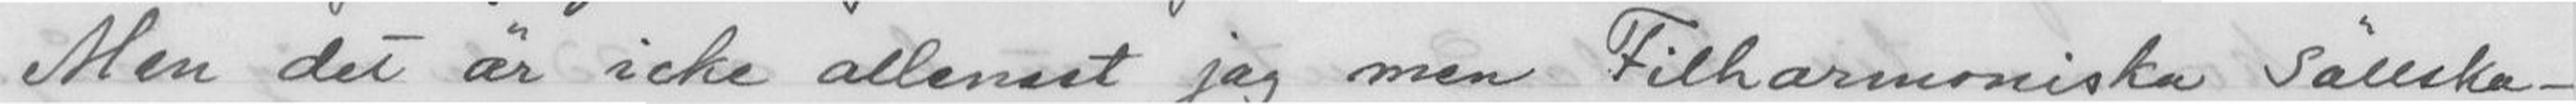Men det är icke allment jag men Filharmoniska Sällska-
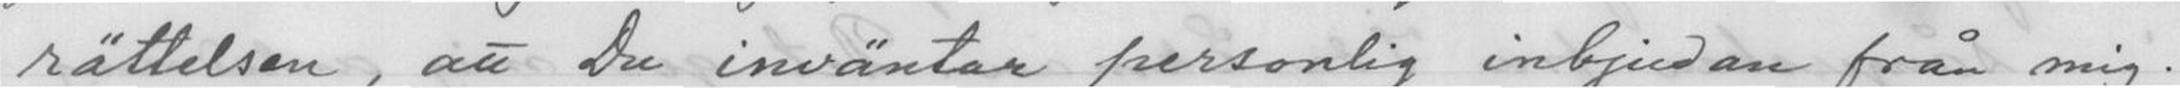rättelsen, att Du inväntar personlig inbjudan från mig.
